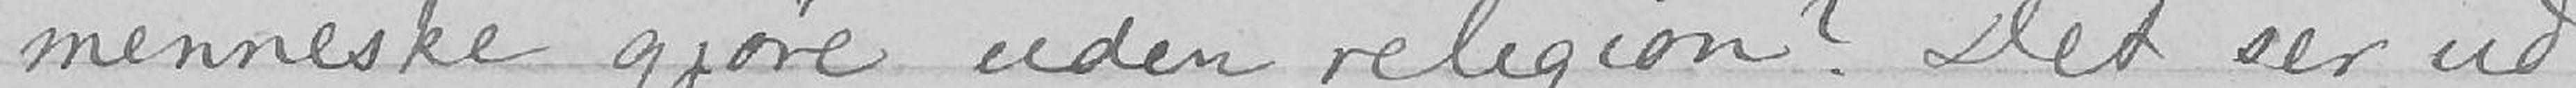menneske gjøre uden religion? Det ser ud
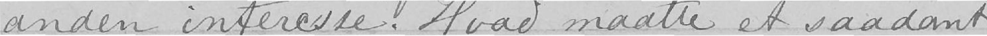anden interesse. Hvad maatte et saadant
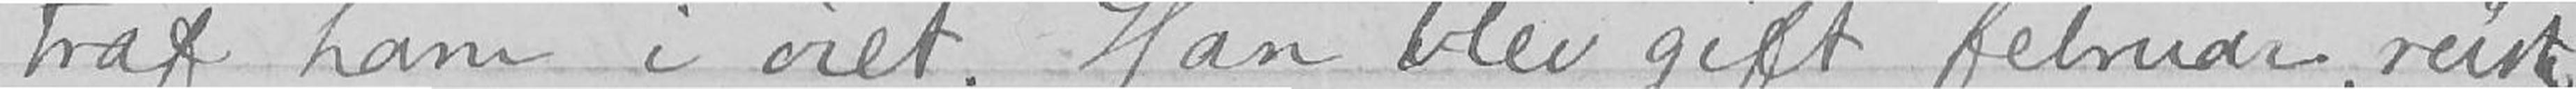traf ham i øiet. Han blev gift februar, reiste
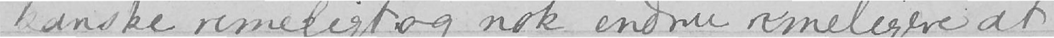kanske rimeligt, og nok endnu rimeligere, at
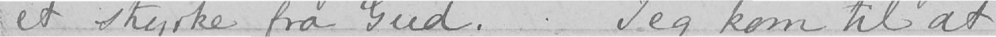et styrke fra Gud. Jeg kom til at
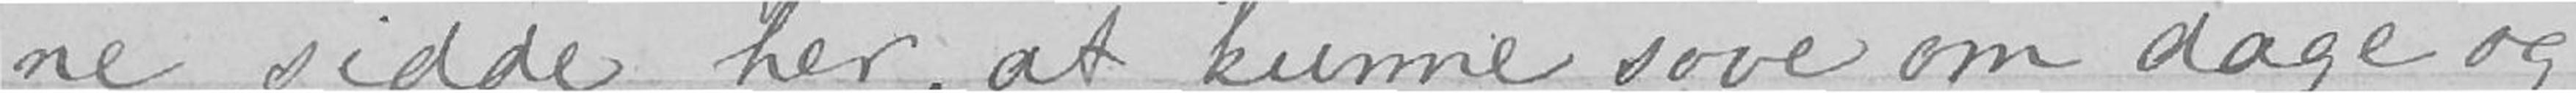ne sidde her, at kunne sove om dage og
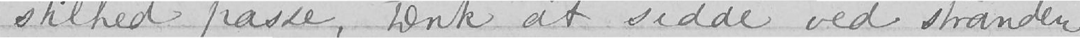stilhed passe, tænk at sidde ved stranden
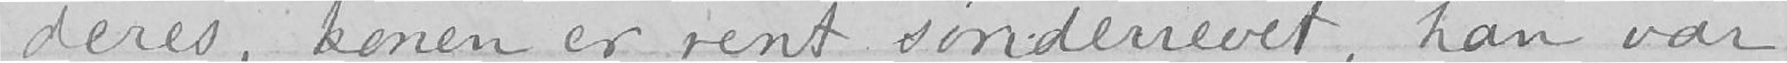deres, konen er rent sønderrevet, han var
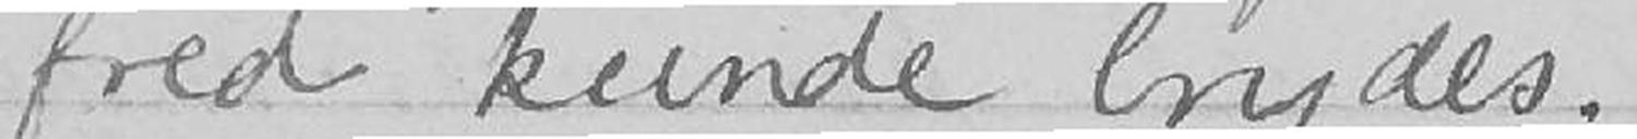fred kunne brydes.
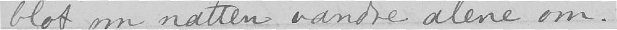blot om natten vandre alene om.
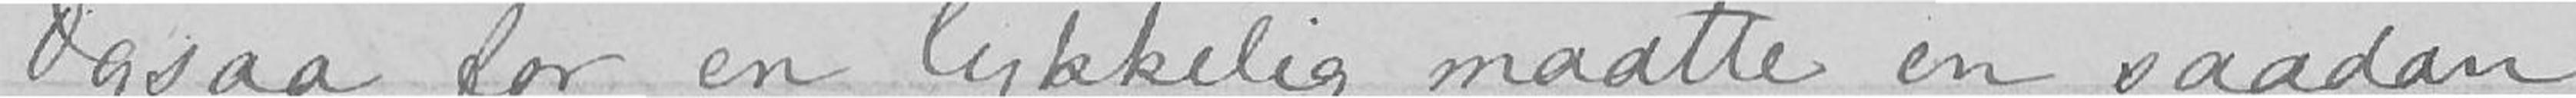Ogsaa for en lykkelig maatte en saadan
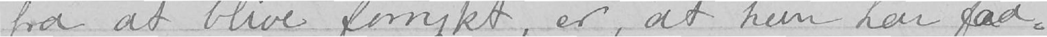fra at blive forrykt, er, at hun har faa-
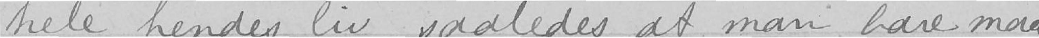hele hendes liv, saaledes at man bare maa
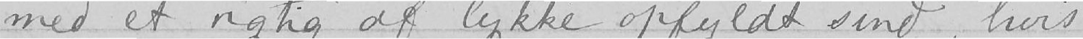med et rigtig af lykke opfyldt sind, hvis
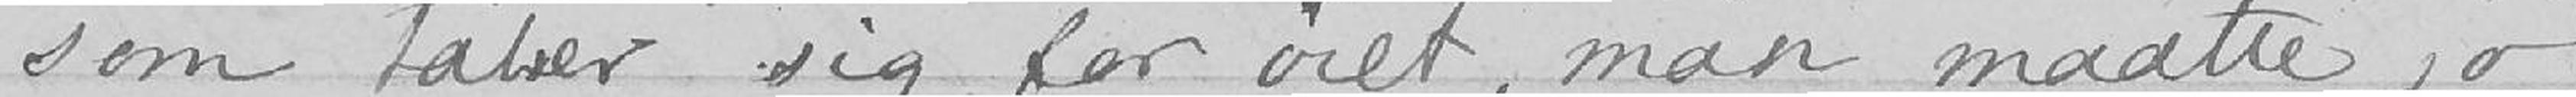som taber sig for øiet, man maatte jo
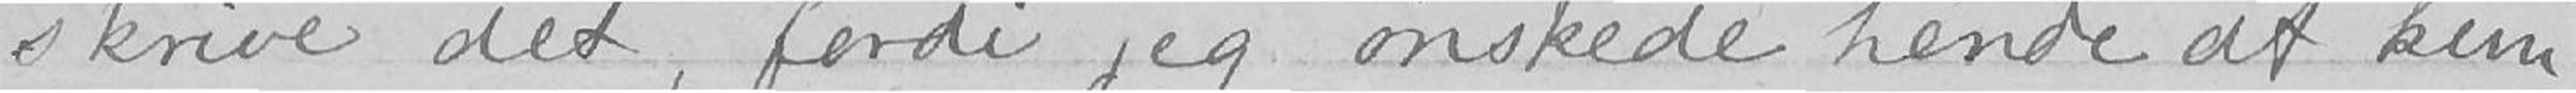skrive det, fordi jeg ønskede hende at kun-
